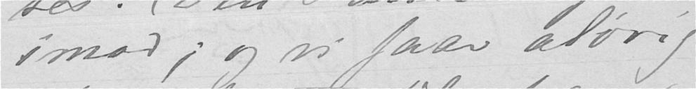imod; og vi faar aldrig
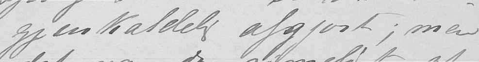gjenkaldelig afgjort; men
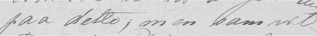paa dette; men samvit-
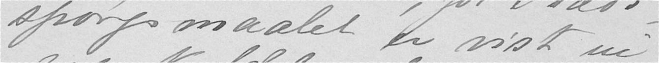spørgsmaalet er vist ui-
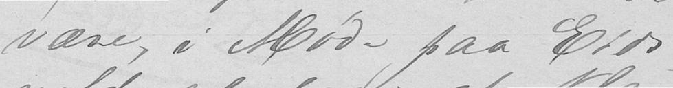være, i Møde paa Eids-
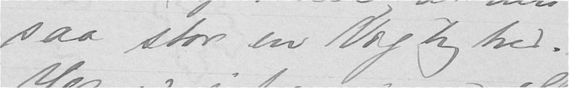saa stor en Vigtighed.
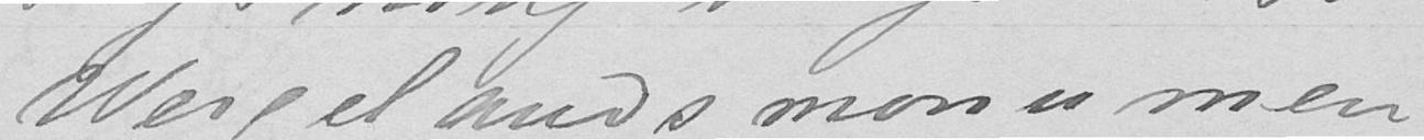Wergelandsmonumen-
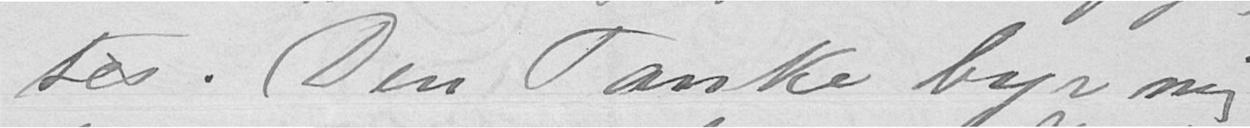tes. Den Tanke byr mig
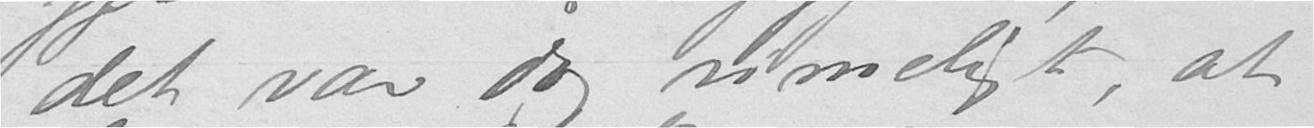det var dog rimeligt, at
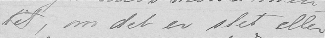tet, om det er slet eller
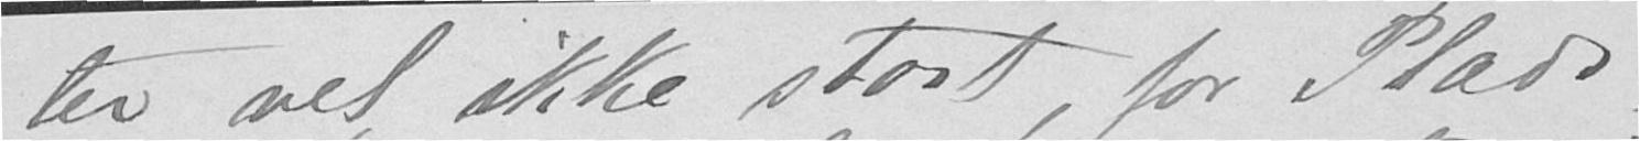ter vel ikke stort, for Plads-
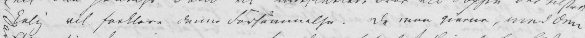kelig vil forklare denne Forsømmelse. De maa gjerne, med den
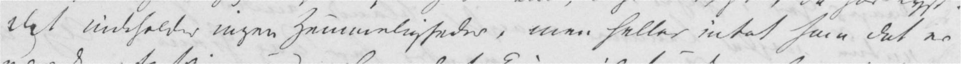Det indeholder ingen Hemmeligheder, men heller intet som det er
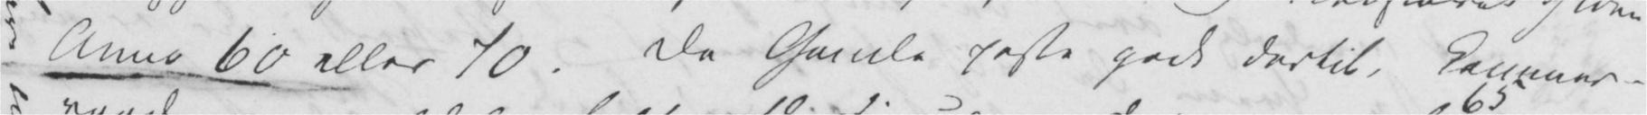Anno 60 eller 70. De Gamle passe godt dertil, Kammer-
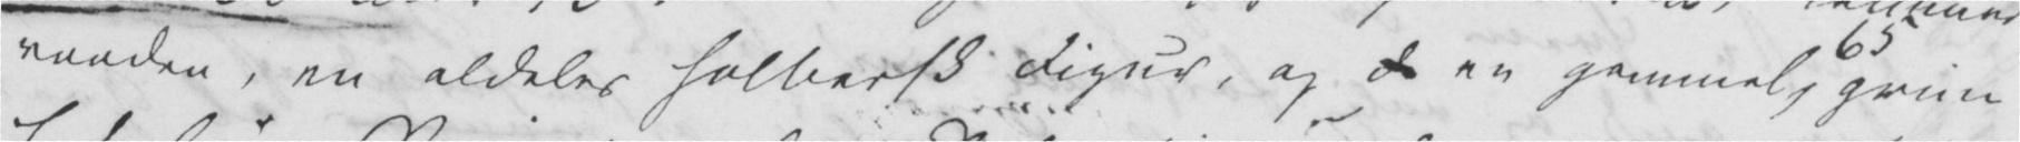raaden, en aldeles holbersk Figur, og d en gammel 65 grim
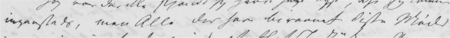ingensteds, men Alle der have bivaanet disse Møder
